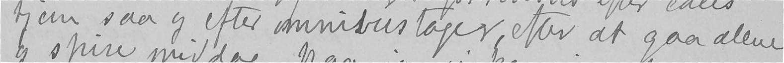hjem saa og efter omnibustoger, efter at gaa alene,
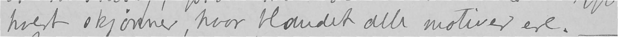hvert skjønner, hvor blandet alle motiver ere. -
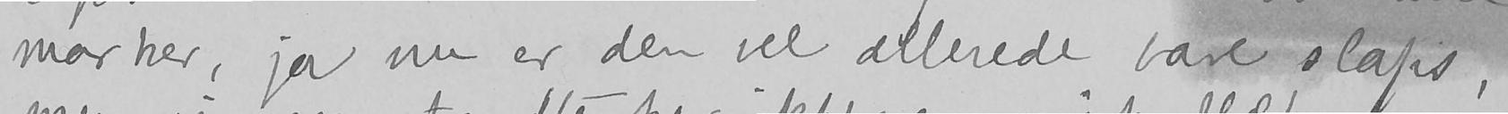marker, ja nu er den vel allerede bare slaps,
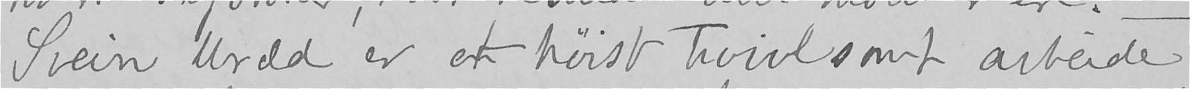Svein Uræd er et høist tvivlsomt arbeide
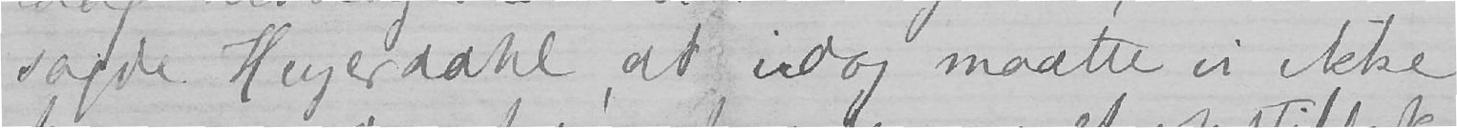sagde Heyerdahl, at idag maatte vi ikke
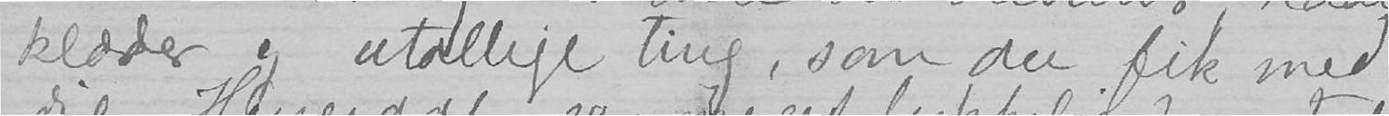klæder og utallige ting, som du fik med
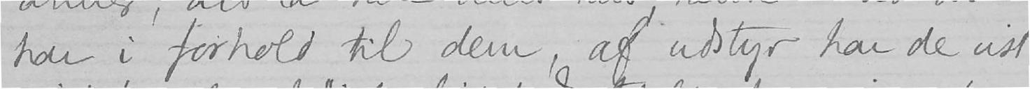har i forhold til dem, af udstyr har de vist
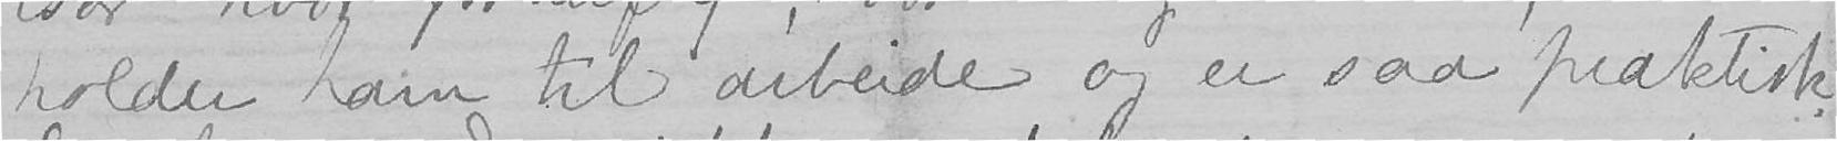holder ham til arbeide og er saa praktisk.
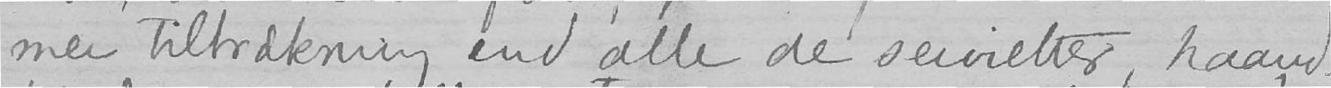mer tiltrækning end alle de servietter, haand-
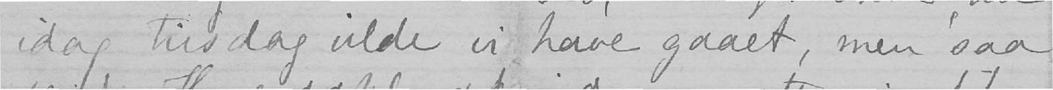idag tirsdag vilde vi have gaaet, men saa
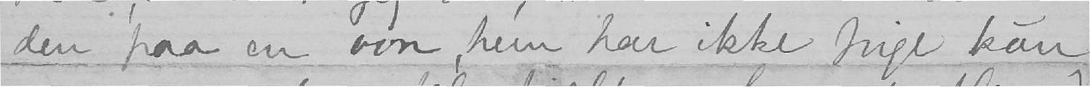den paa en ovn, hun har ikke pige kun
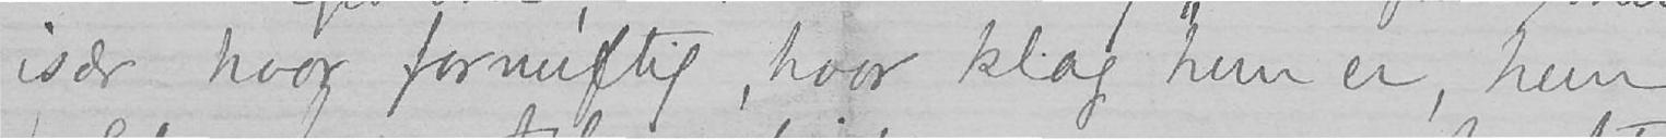især hvor fornuftig, hvor klog hun er, hun
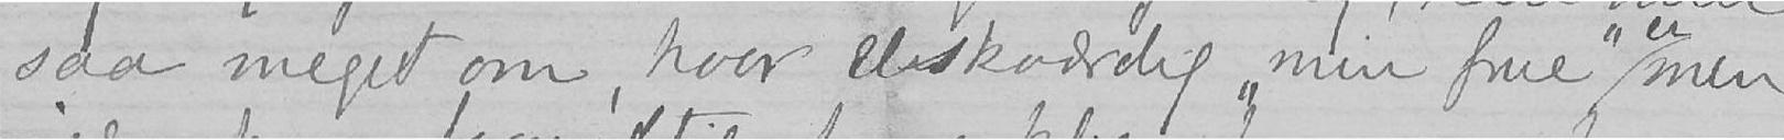saa meget om, hvor elskværdig «min frue» er, men
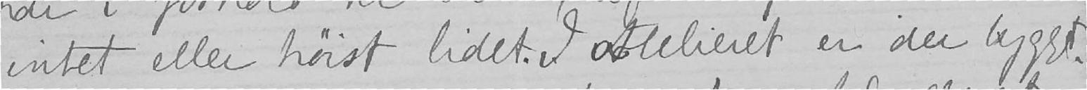intet eller høist lidet. I Aatelieret er der bygget
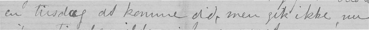en tirsdag at komme did, men gik ikke, nu
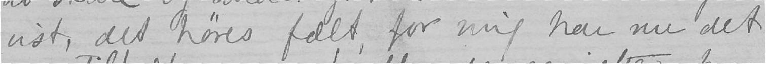vist, det høres fælt, for mig har nu det
